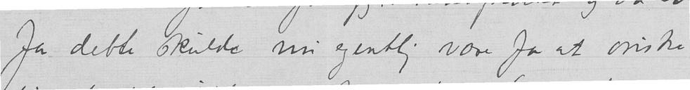Ja dette skulde nu egentlig være for at ønske
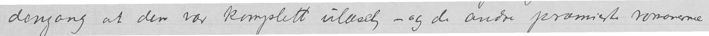dengang at den var komplett ulæselig – og de andre præmierte romanerne
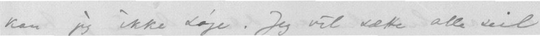kan jeg ikke søge. Jeg vil sætte alle seil
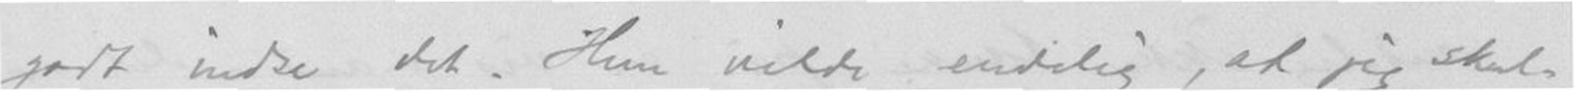godt indse det. Hun vilde endelig, at jeg skul-
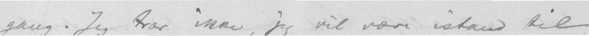gang. Jeg tror ikke, jeg vil være istand til
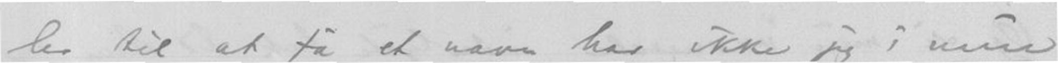ler til at få et navn har ikke jeg i min
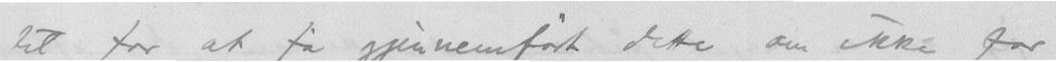til for at få gjennemført dette om ikke for
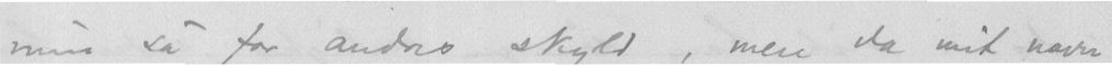min så for andres skyld, men da mit navn
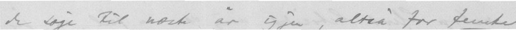de søge til næste år igjen, altså for femte
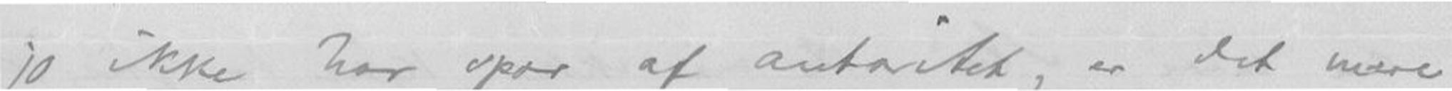jo ikke har spor af autoritet, er det mere
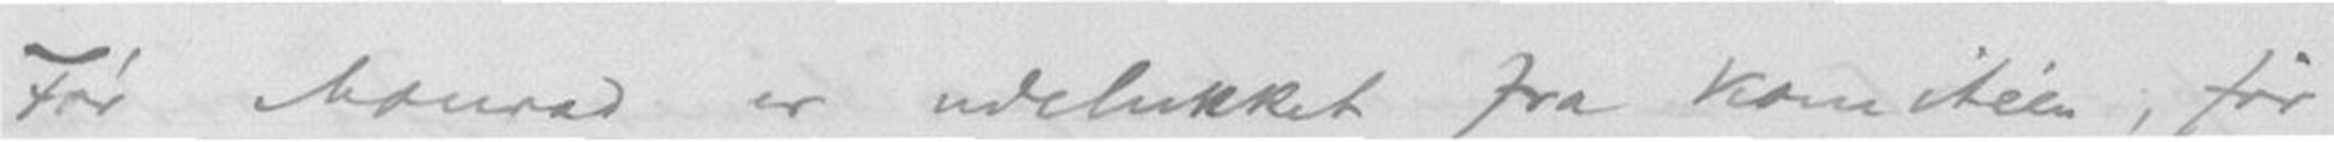Før Monrad er udelukket fra komitèen, før
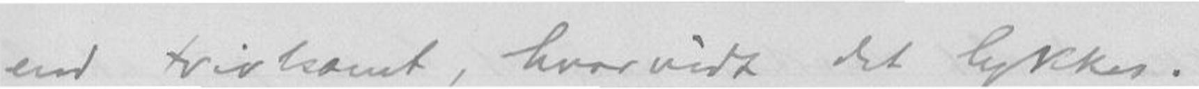end tvivlsomt, hvorvidt det lykkes.
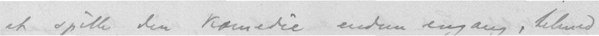at spille den komedie endnu engang, tilmed
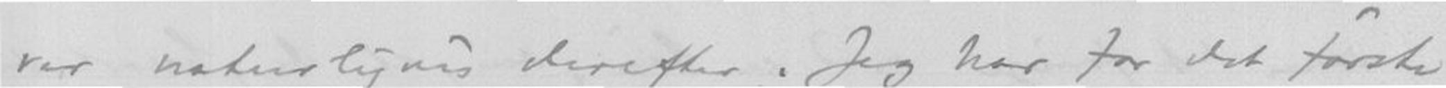ver naturligvis derefter. Jeg har for det første
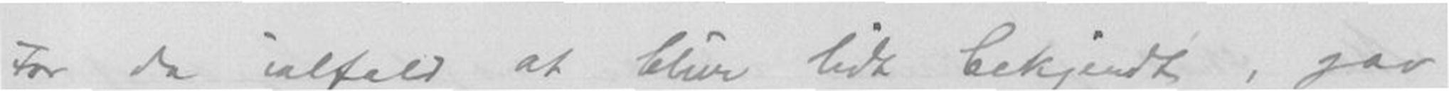For da ialfald at blive lidt bekjendt, gav
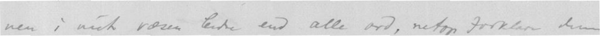vere i mit væsen bedre end alle ord, netop forklare dem
